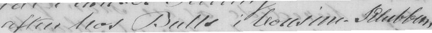aften hos Bulls i Cousine-Klubben
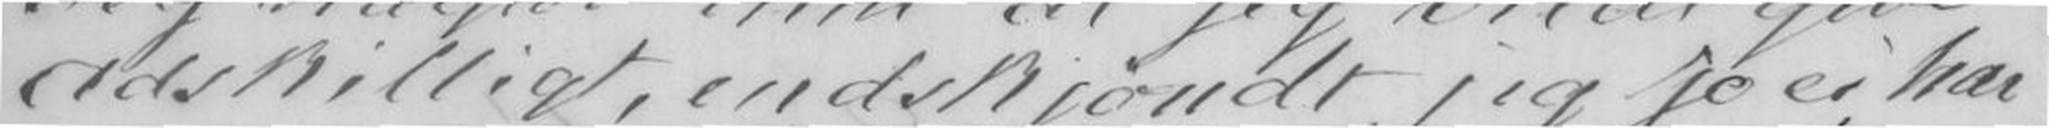adskilligt, endskjøndt jeg jo ei har
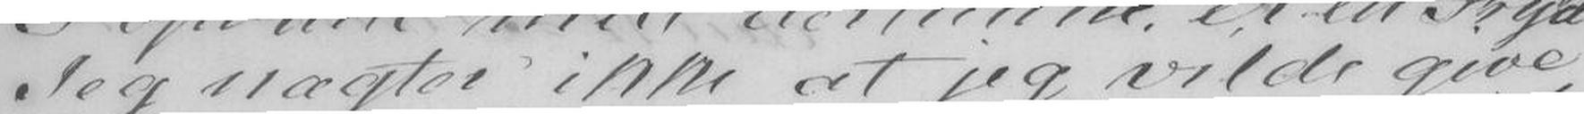Jeg nægter ikke at jeg vilde give
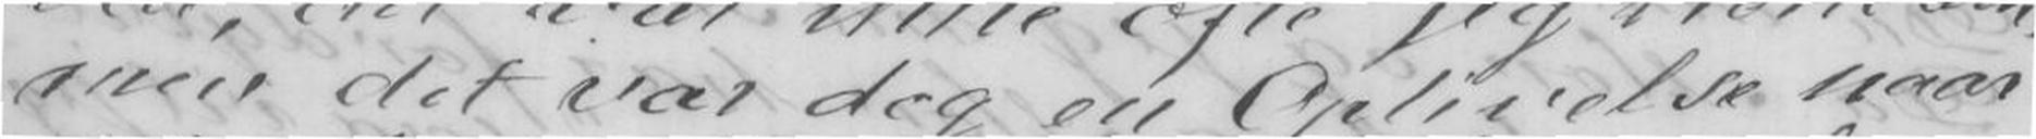men det var dog en Oplevelse naar
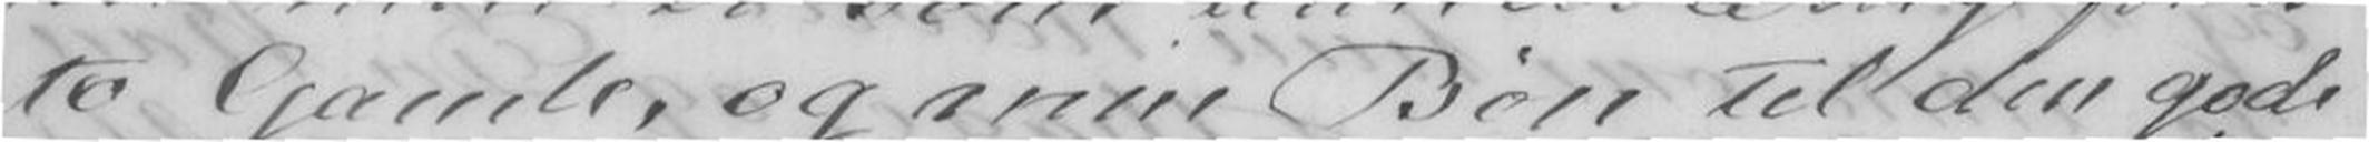to Gamle, og min Bøn til den gode
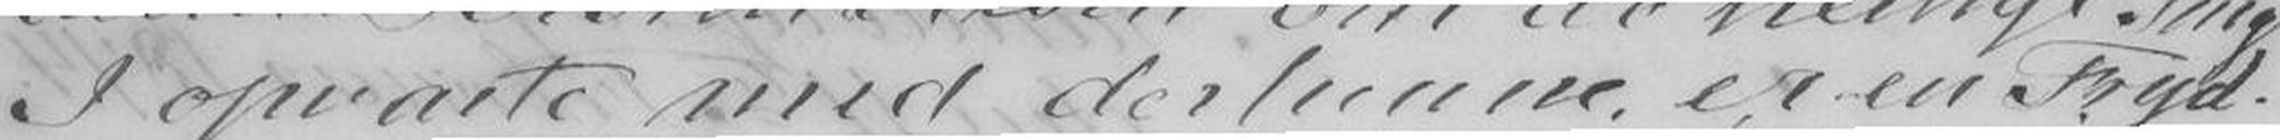I opvarte med derhenne er en Fryd.
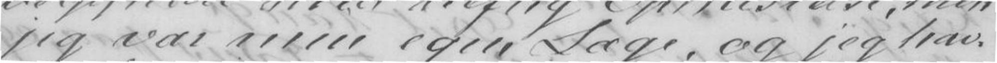jeg var min egen Læge, og jeg hav-
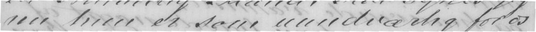nu hun er som uunværlig for os
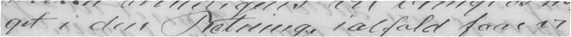get i den Retning ialfald faar vi
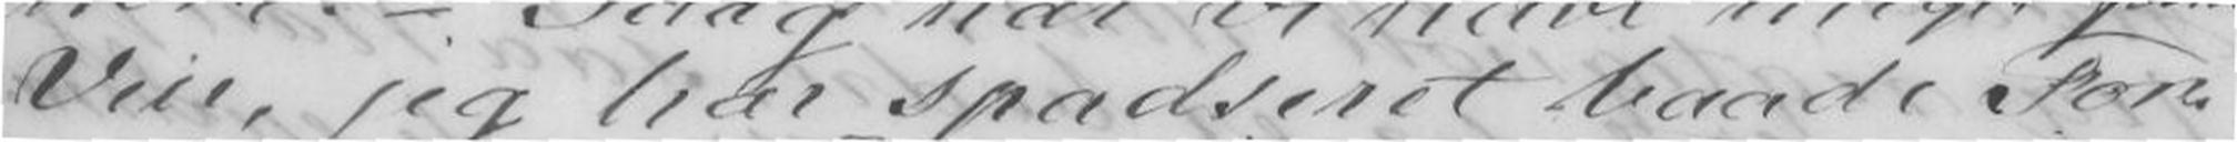Veir, og jeg har spadseret baade For-
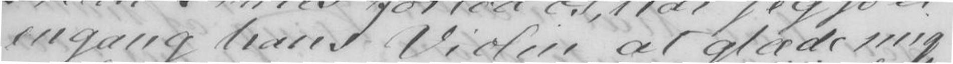engang hans Violin at glæde mig
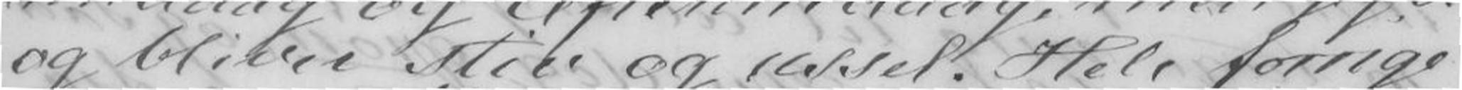og bliver stiv og ussel. Hele forrige
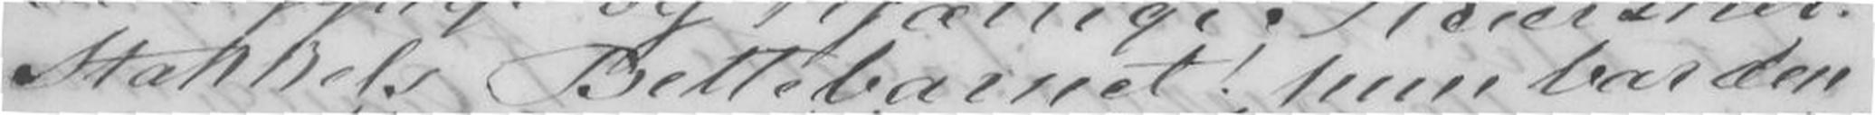Stakkels Bettebarnet! hun bar den
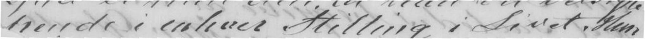hende i enhver Stilling i Livet. Hun
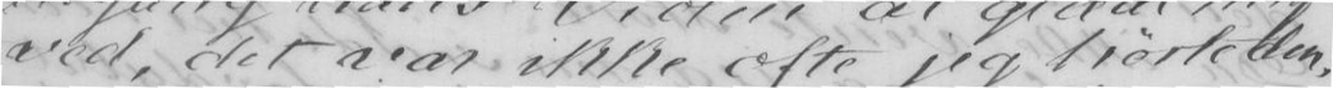ved, det var ikke ofte jeg hørte den,
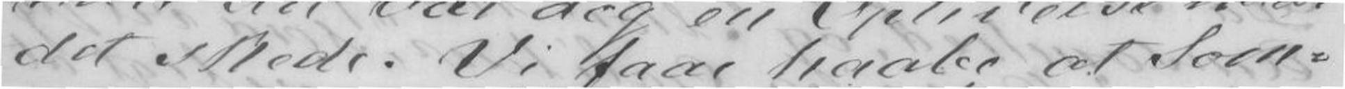det skede. Vi faar haabe at Som-
